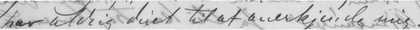har aldrig duet til at anerkjende mig.
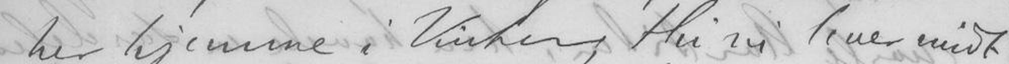her hjemme i Vinter; thi vi lever midt
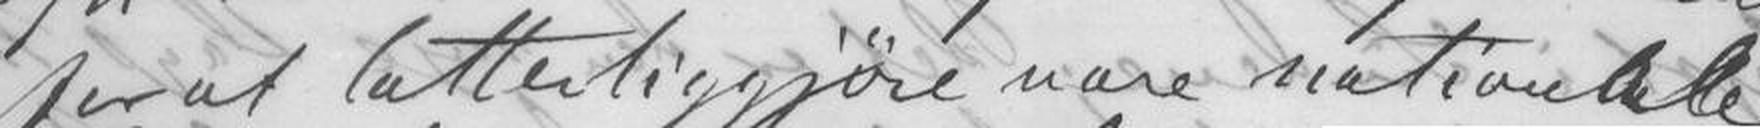for at lattergjøre vore nationale
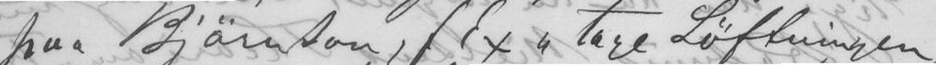paa Bjørnson, f. Ex tage Løftningen
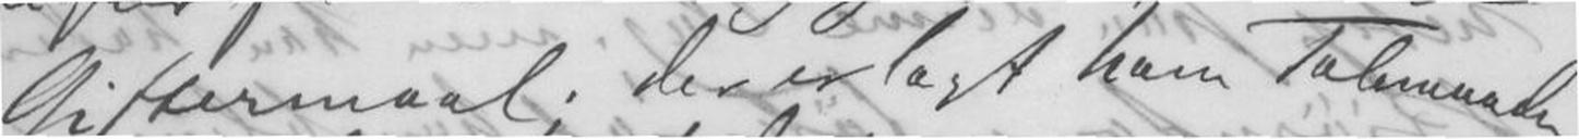Giftermaal; der er lagt ham Talemaaden
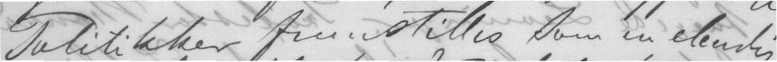Politikker fremstilles som en elendig
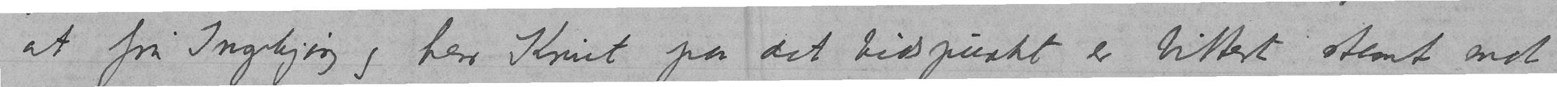at fru Ingebjørg og herr Knut paa det tidspunkt er bittert stemt mot
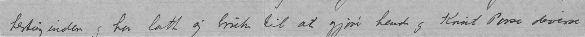hertuginden og har latt sig bruke til at gjøre hende og Knut Porse diverse
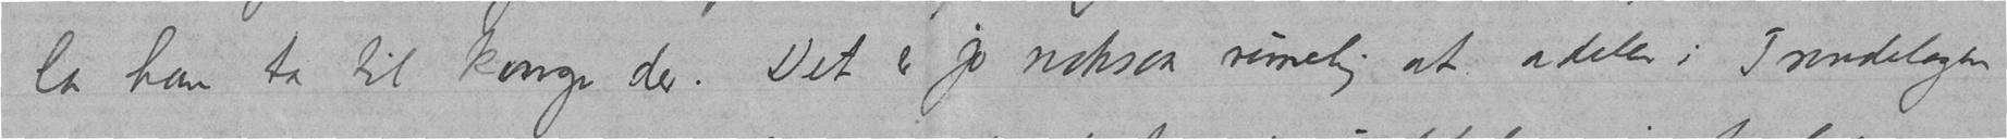la han ta til konge der. Det er jo noksaa rimelig at adelen i Trøndelagen
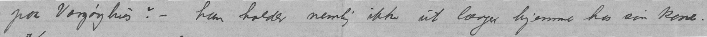paa Vargøyhus? – han holder nemlig ikke ut længer hjemme hos sin kone.
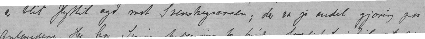er blit flyttet syd mot Svenskegrænsen; der var jo en del gjøring paa
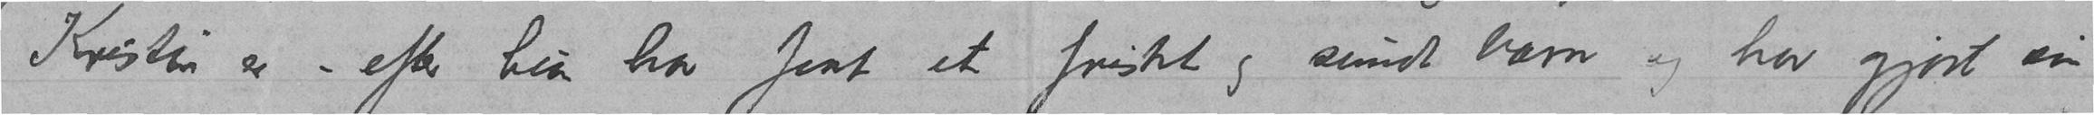Kristin er – efter hun har faat et friskt og sundt barn og har gjort sin
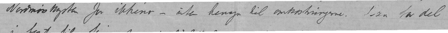Nordmørskysten for ikkeno – uten hensyn til omkostningerne. Han tar del
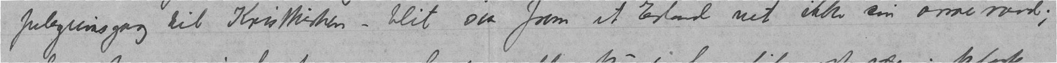pilegrimsgang til Kristkirken – blit saa from at Erlend vet ikke sin arme raad;
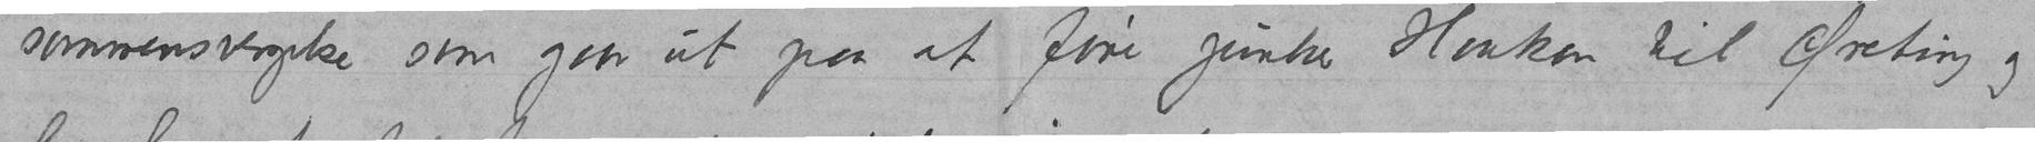sammensvergelse som gaar ut paa at føre junker Haakon til Øreting og
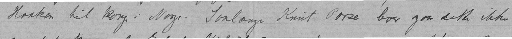Haakon til konge i Norge. Saalænge Knut Porse lever gaar dette ikke
